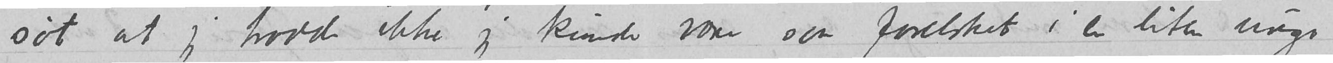søt at jeg trodde ikke jeg kunde være saa forelsket i en liten unge
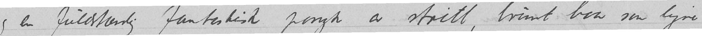og en fuldstændig fantastisk paryk av stritt, brunt haar som ligner
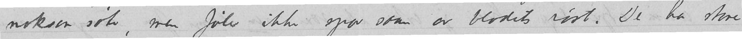noksaa søte, men føler ikke spor saan av blodets røst.  De har store
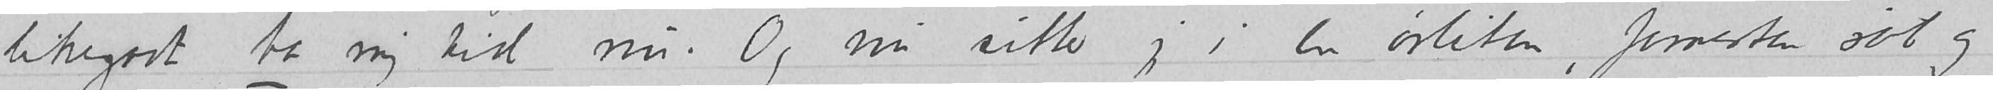likegodt ta   mig tid nu.  Og nu sitter jeg i en ørliten, forresten søt og
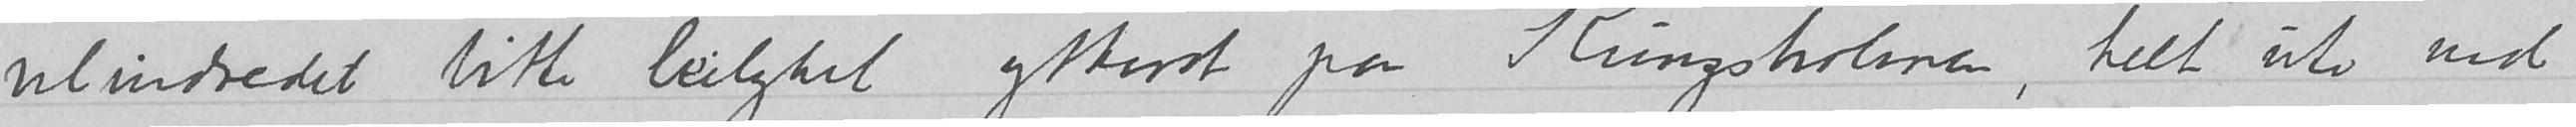velindredet liten leilighet ytterst paa Kungsholmen, helt ute ved
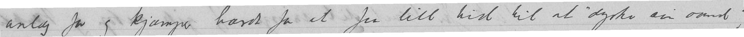anlæg for og kjæmper hardt for at faa litt tid til at «dyrke sin aand»,
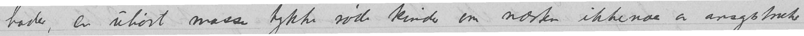hoder, en uhørt masse tykke røde kinder om næsten ikkenoe av ansigtstræker
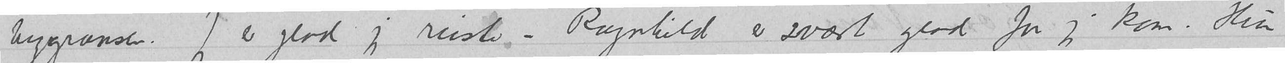bygrænsen.  Jeg er glad jeg reiste – Ragnhild er svært glad for jeg kom.   Hun
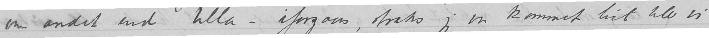om andet end Ulla –  iforgaars, straks jeg var kommet hit blev vi
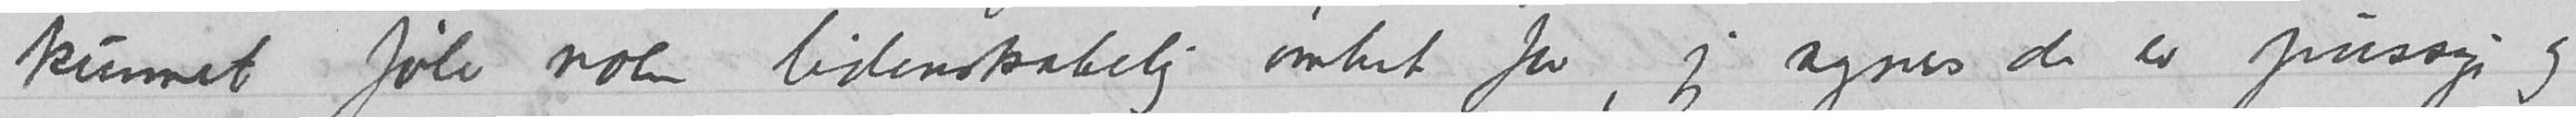kunnet føle noe lidenskabelig ømhet for, jeg synes de er pussige og
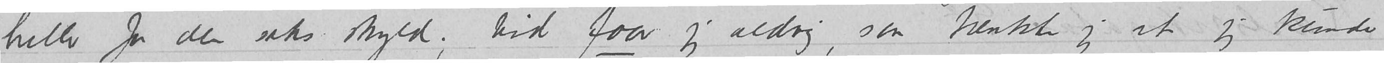heller for den saks skyld; tid faar jeg aldrig, saa tænkte jeg at jeg kunde
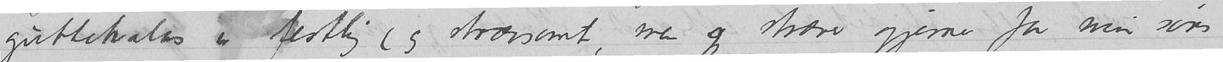guttekalas er festlig (og strævsomt men jeg stræver gjerne for min søns
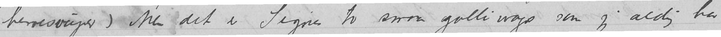herresouper)  Men det er Signes to smaa gullunger som jeg aldrig har
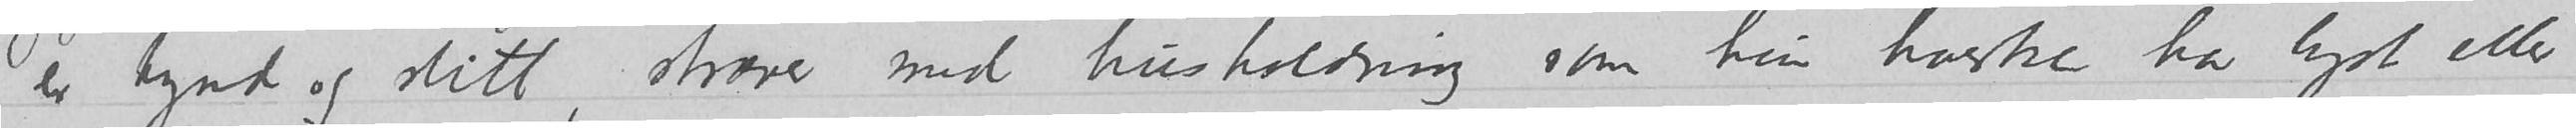er tynd og slitt, stræver med husholdning som hun hverken har lyst eller
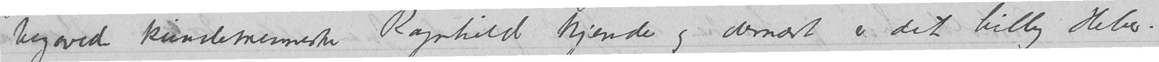begavede kvindemenneske Ragnhild kjender og dernest er det Lilly Heber.
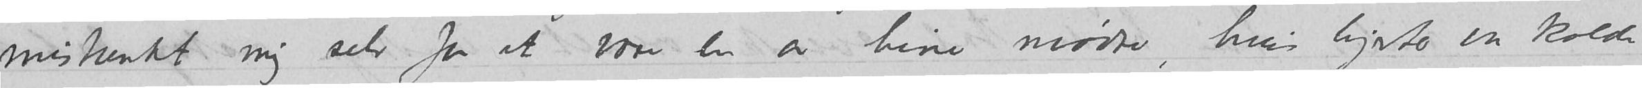mistænkt mig selv for at være en av hine mødre, hvis hjerter var kolde
